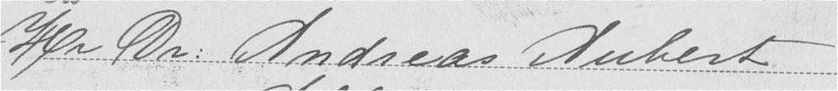Hr Dr Andreas Aubert
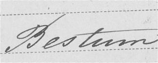Bestum
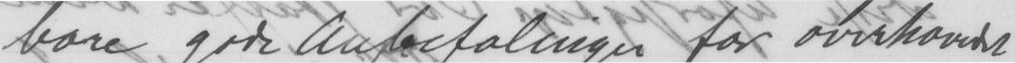bare gode Anbefalinger for overhodet
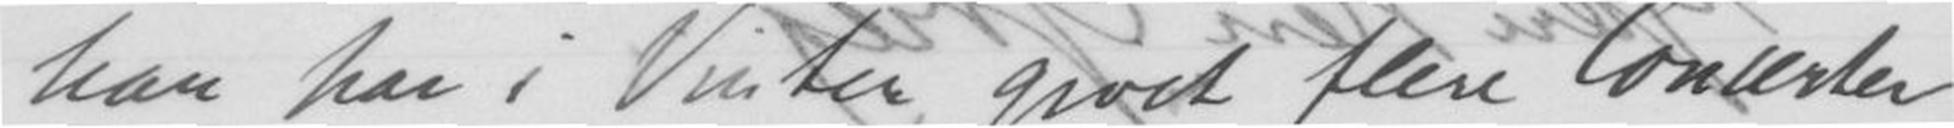han har i Vinter givet flere Concerter
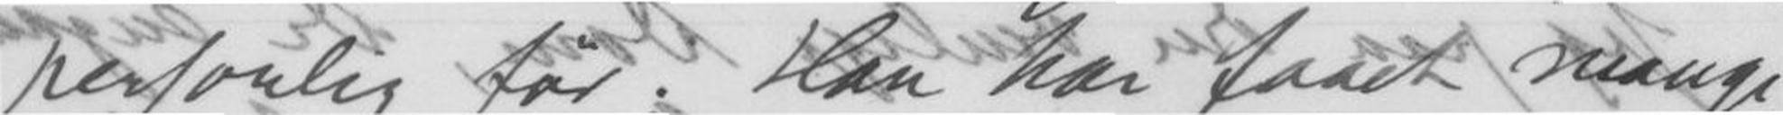personlig før. Han har faaet mange
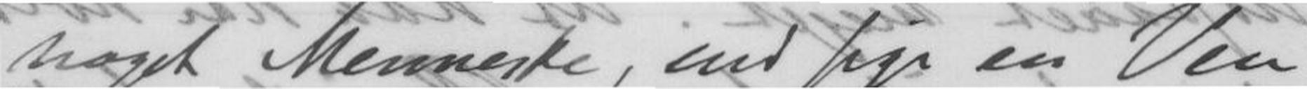nogit Menneske, end sige en Ven
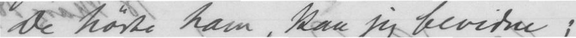De hørte ham, kan jeg bevidne;
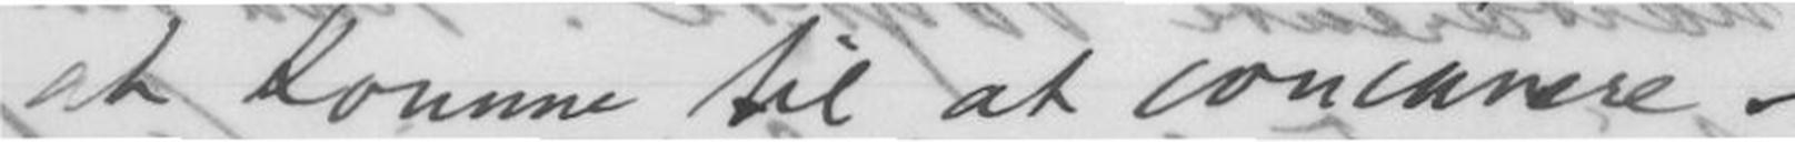at Komme til at Concurere-
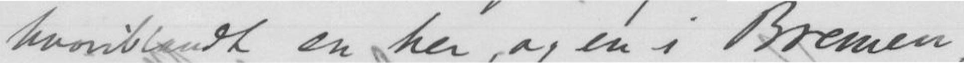hvoriblandt en her og en i Bremen,
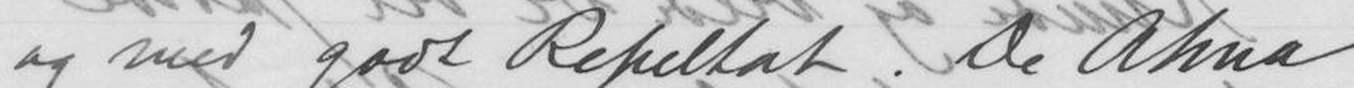og med godt resultat. De Ahna
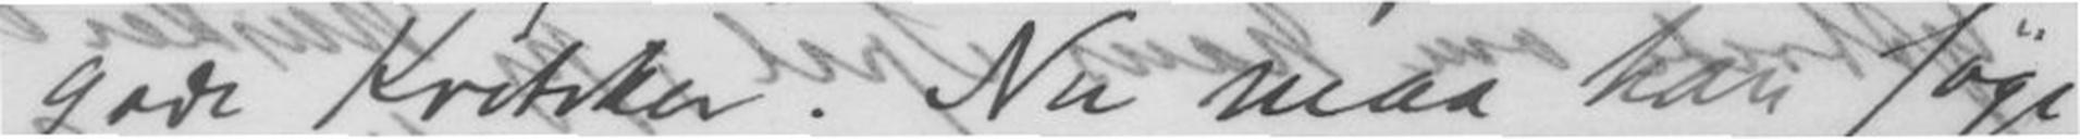gode Kritiker. Nu maa han søge
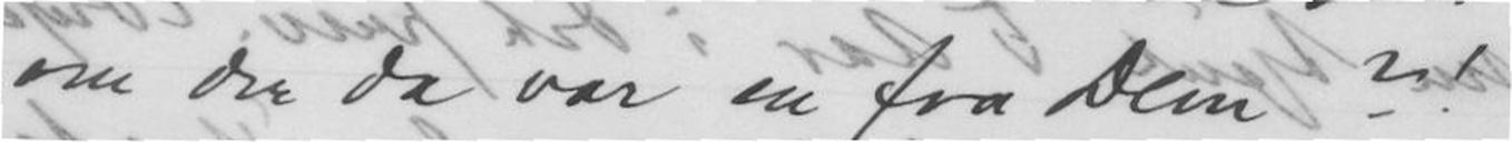om der da var en fra Dem?!
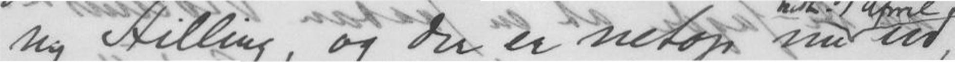ny Stilling, og der er netop nu ud-
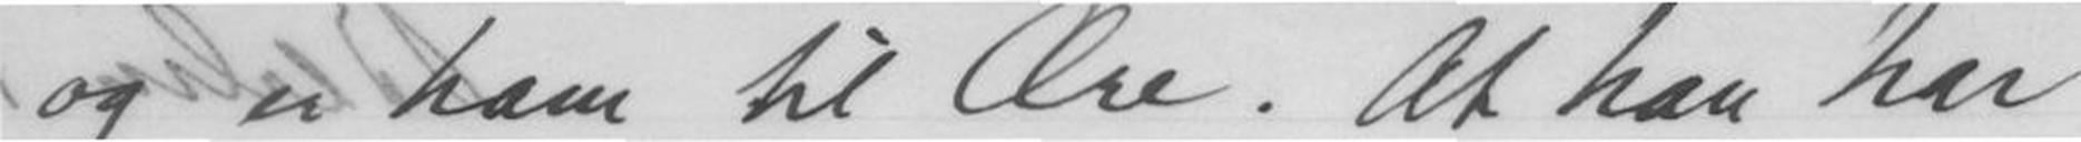og er ham til Ære. At han har
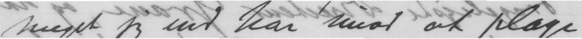meget jeg end har imod at plage
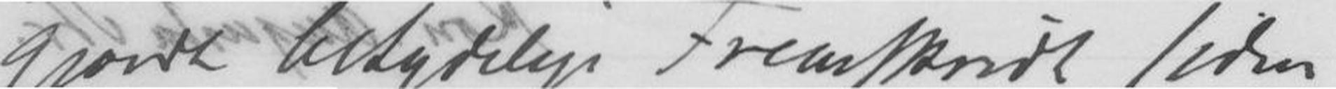gjordt betydelige Fremskridt siden
